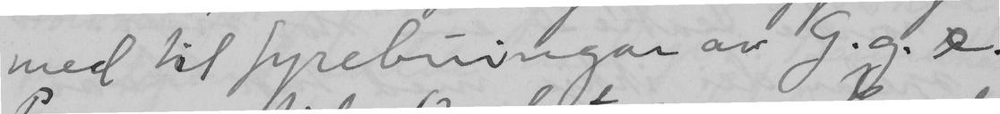med til fyrebuingar av J.g.e.
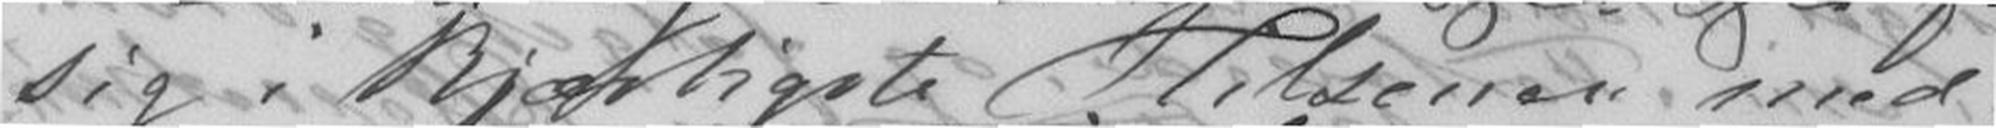sig kjærligste Hilsener med
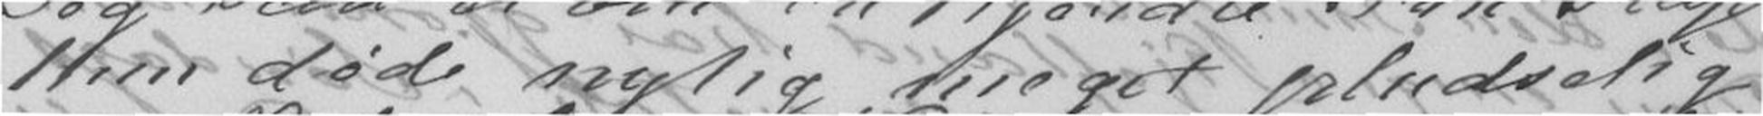Hun døde nylig meget pludselig
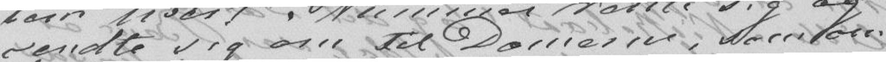vendte sig om til Damerne, som om
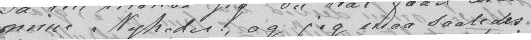mine Nyheder, og jeg maa saaledes
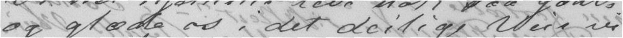0g glæde os i det deilige Veir vi
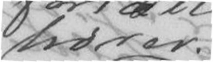hører.
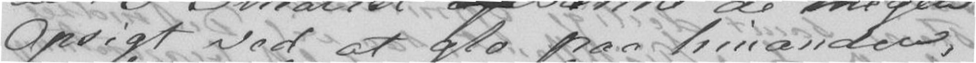Opsigt ved at glo paa hinanden,
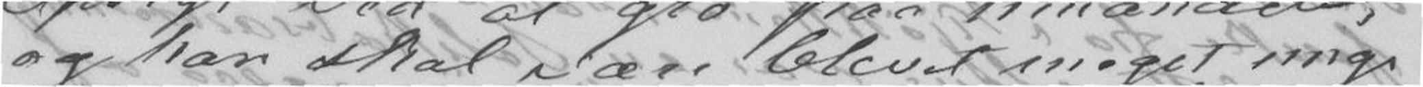og han skal være blevet meget ung-
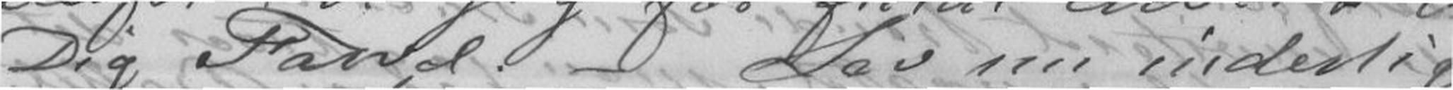Dig Farvel - Lev nu inderlig
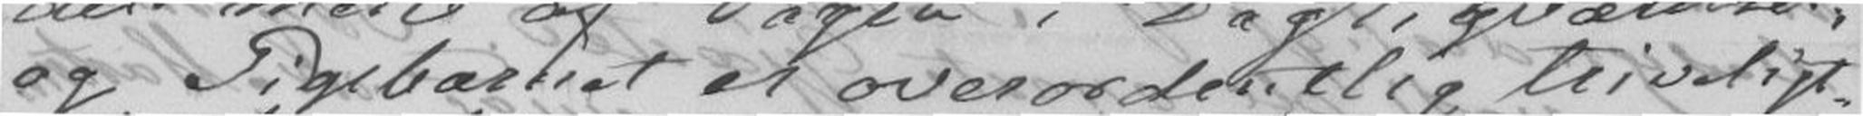og Pigebarnet er overordentlig triveligt.
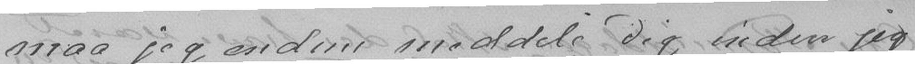maa jeg endnu meddele Dig inden jeg
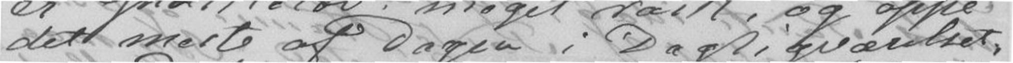det meste af dagen i Dagligværelset,
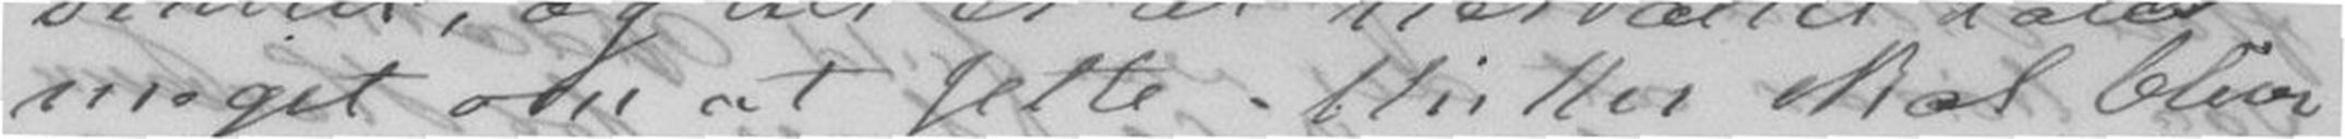meget om at Jette Müller skal blive
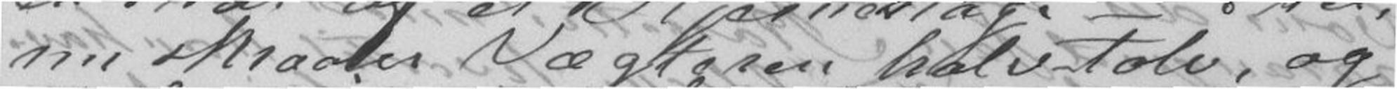nu skraaler Vægteren halv tolv, og
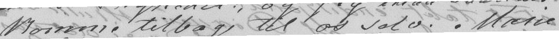komme tilbage til os selv. Marie
